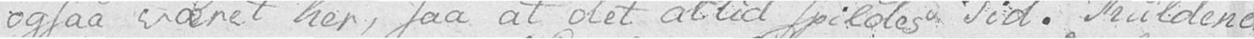ogsaa været her, saa at det altid spildes Tid. Kulden e
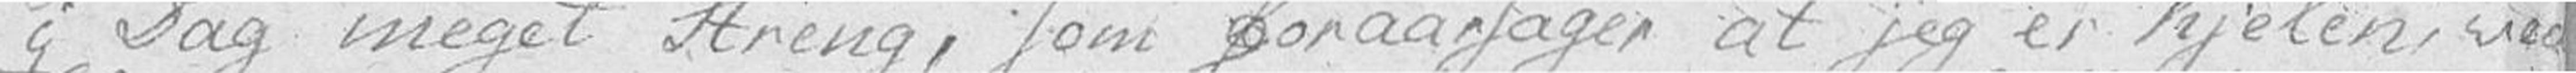i Dag meget Streng, som foraarsager at jeg er Kjelen, ved
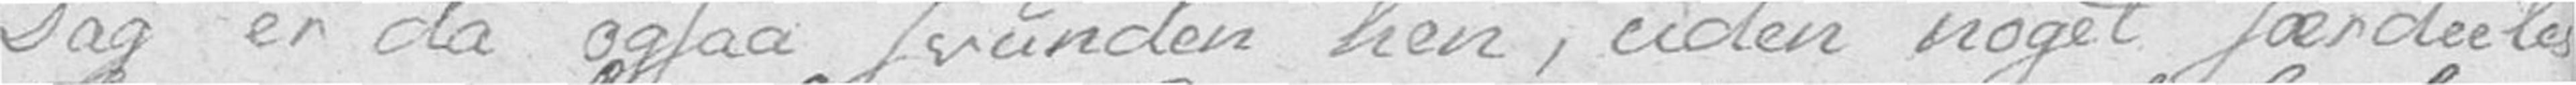Dag er da ogsaa svunden hen, uden noget særdeeles
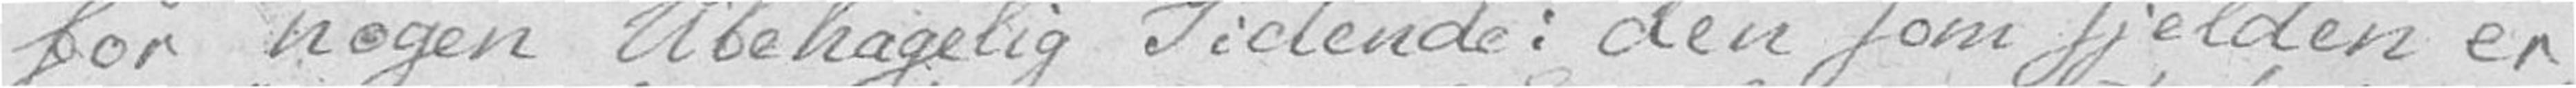for nogen Ubehagelig Tidende: den som sjelden er
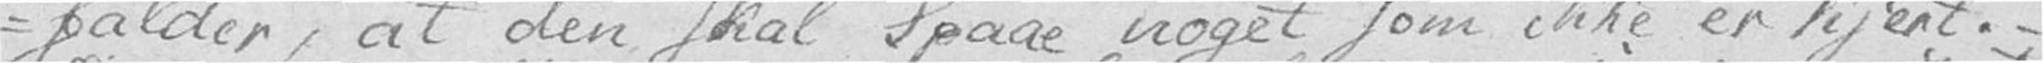-falder, at den skal Spaae noget som ikke er Kjert. –
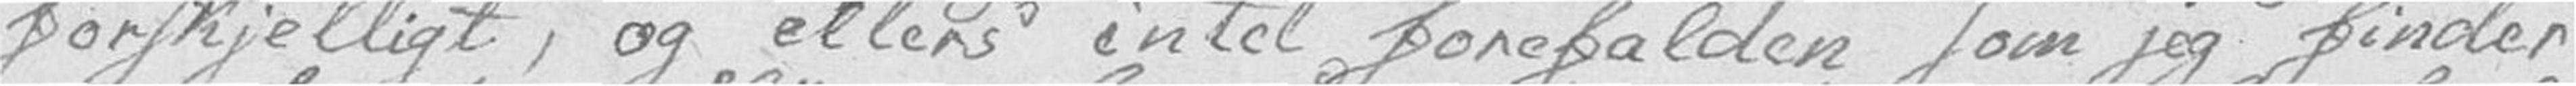forskjelligt, og ellers intet forefalden som jeg finder
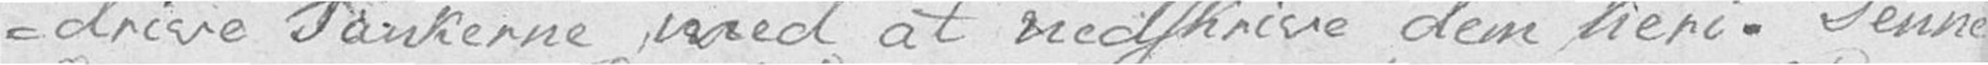-drive Tankerne med at nedskrive dem heri. Denne
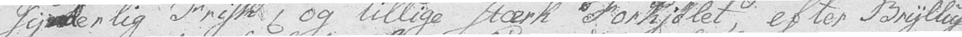synderlig Frisk, og tillige stærk Forkjølet, efter Bryllup
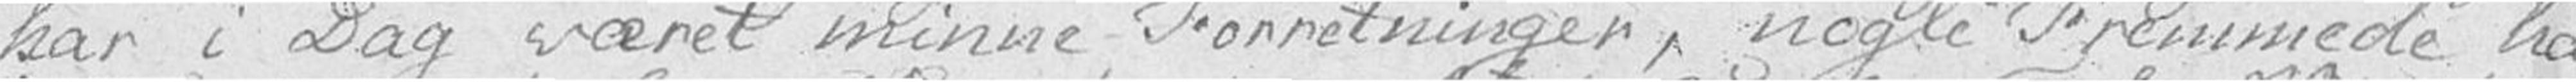har i Dag været minne Forretninger, nogle Fremmede ha
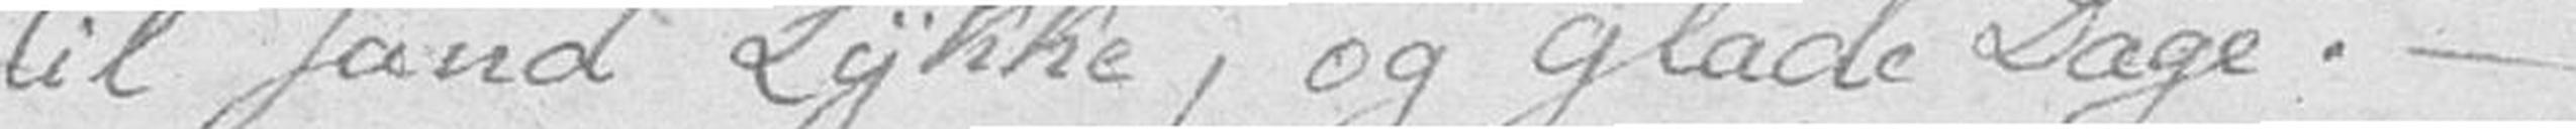til sand Lykke, og glade Dage. –
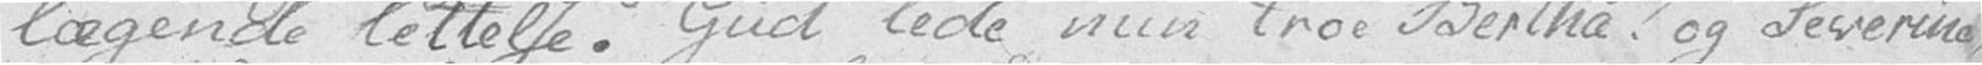lægende lettelse. Gud lede min troe Bertha! og Severine,
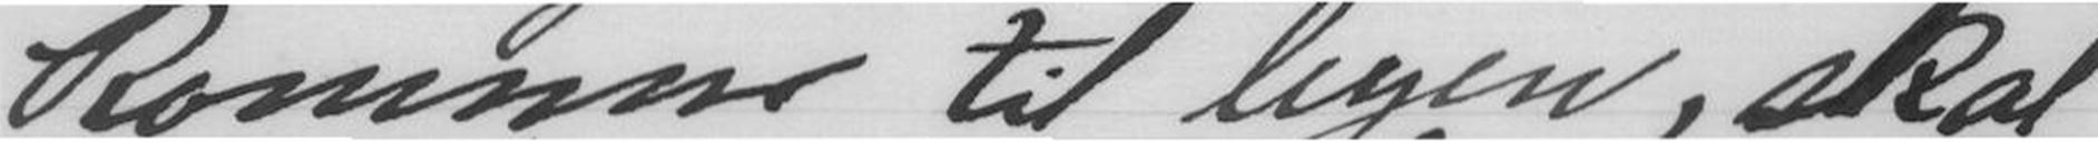kommer til byen, skal
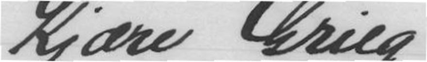Kjære Grieg
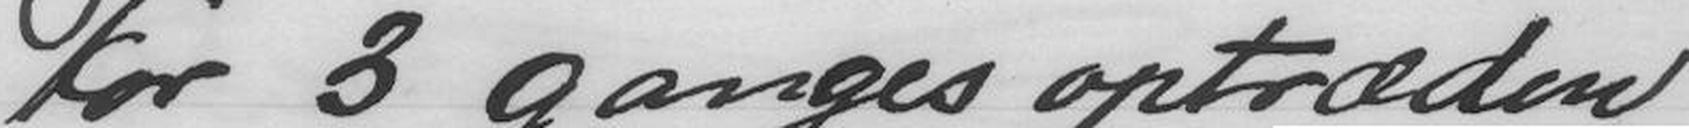For 3 ganges optræden
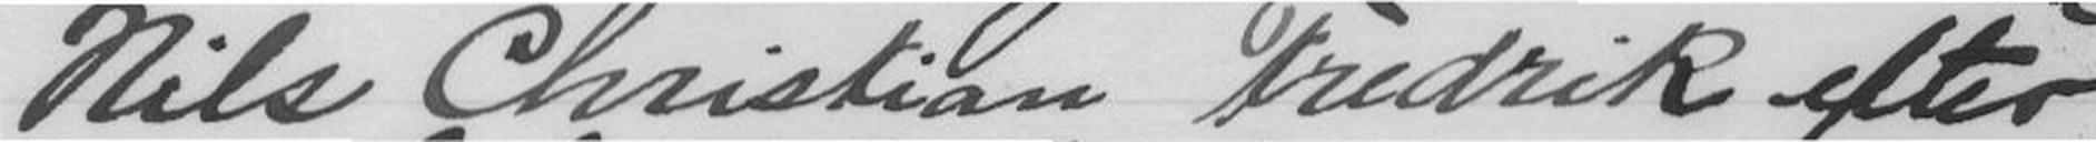Nils Christian Fredrik efter
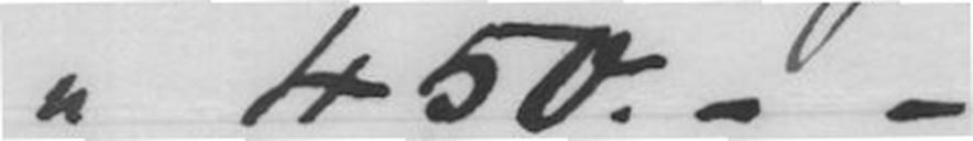" 450,- -
